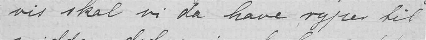vis skal vi da have ryper til
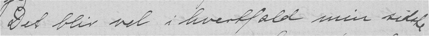Det blir vel i hvertfald min sidste
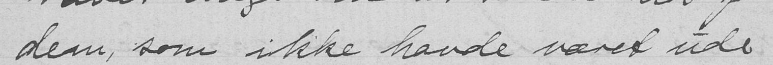dem, som ikke havde været ude
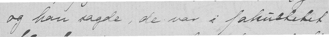og han sagde, de var i fakultetet
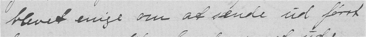blevet enige om at sende ud først
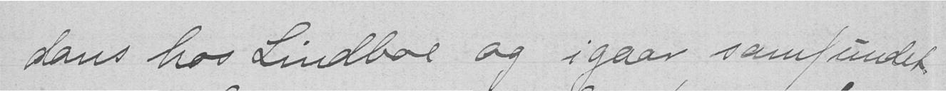dans hos Lindboe og igaar samfundet,
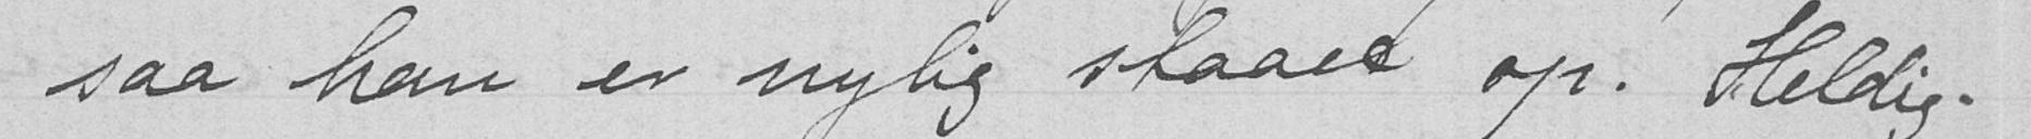- saa han er nylig staaet op. Heldig-
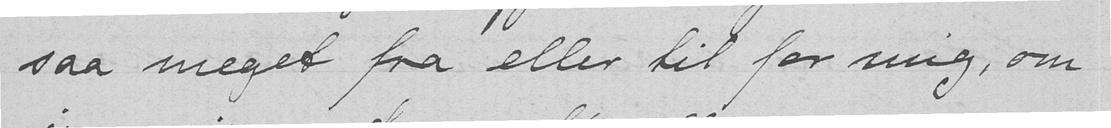saa meget fra eller til for mig, om
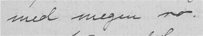med megen ro.
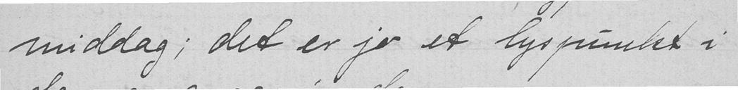middag; det er jo et lyspunkt i
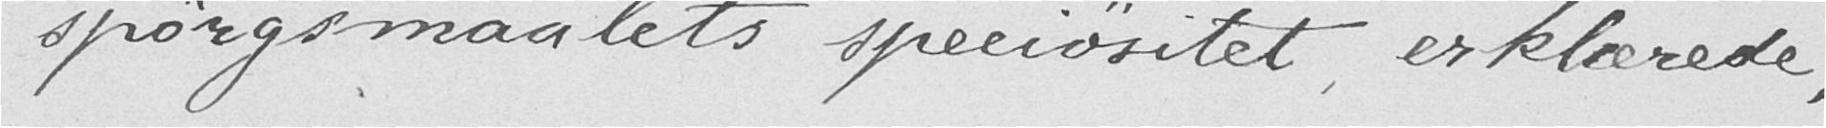spørgsmaalets speciøsitet, erklærede,
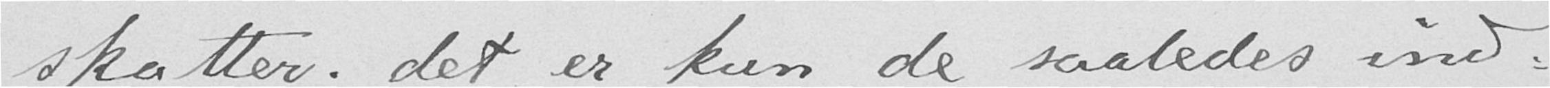skatter; det er kun de saaledes ind-
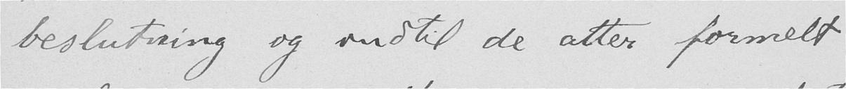beslutning og indtil de atter formelt
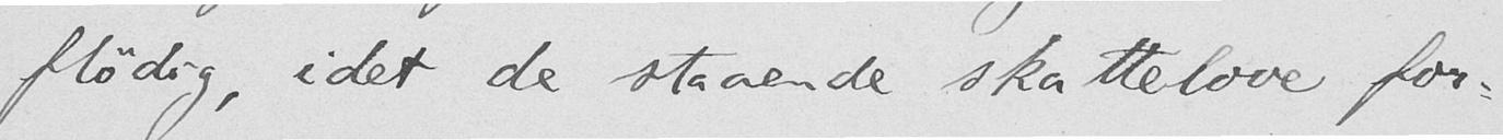flødig, idet de staaende skattelove for-
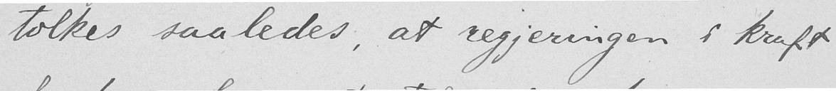tolkes saaledes, at regjeringen i kraft
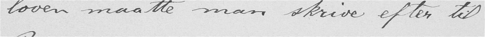loven maatte man skrive efter til
